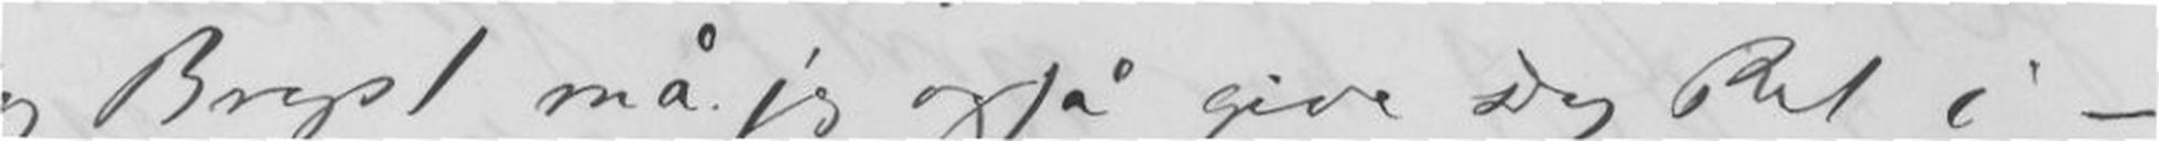og Bryst må jeg også give Ret i -
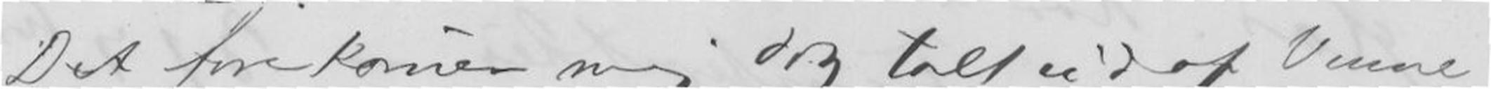Det forekommer mig dog talt udaf Venne
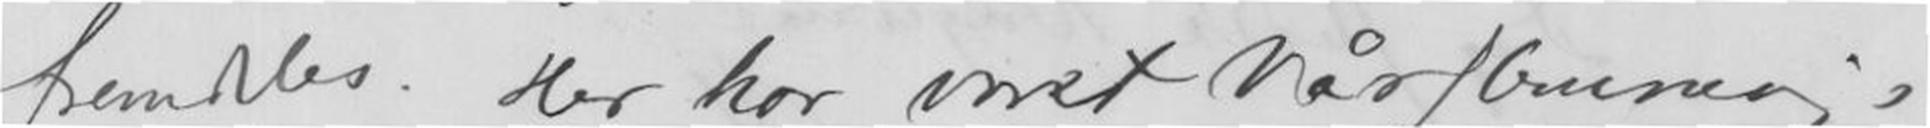fremdeles. Her har været Vårstemnings
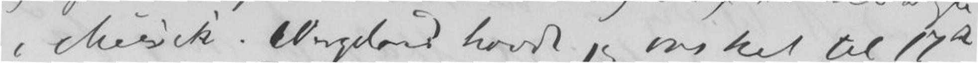i Musik. Wergeland havde jeg ønsket til 17de
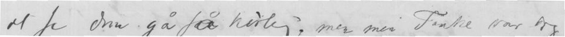at se dem gå så hurtig, men min Tanke var dog
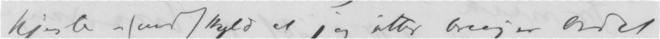hjerte -(undskyld at jeg atter bringer Ordet
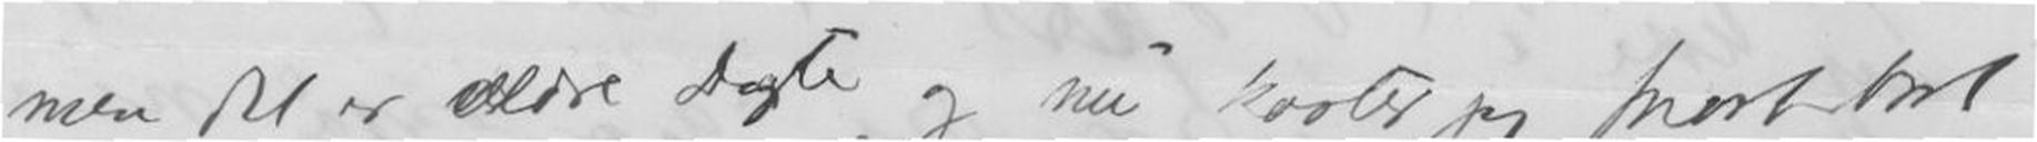men det er Ældre Digte og nu kaster jeg snart bort
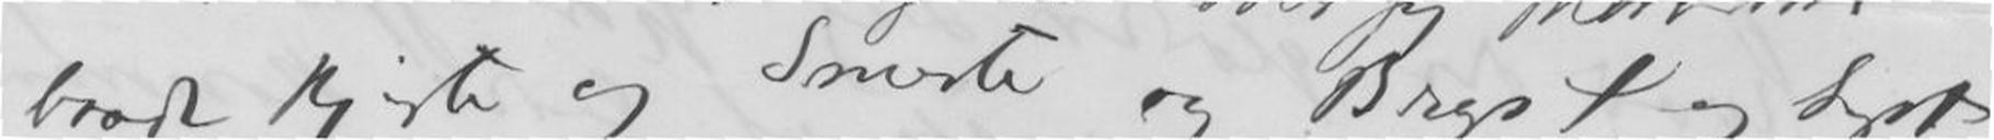baade Hjerte og Smerte og Bryst og Lyst
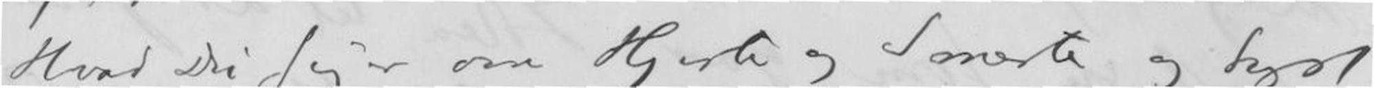Hvad Du siger om Hjerte og Snerte og Lyst
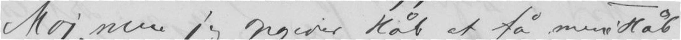Maj, men jeg opgiver Håb at få mine Håb
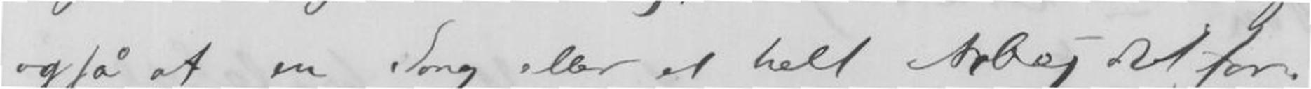også at en Song eller et helt Arbejdet far-
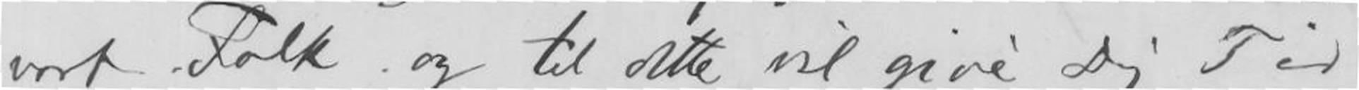vort Folk og til sette vil give Dig Tid
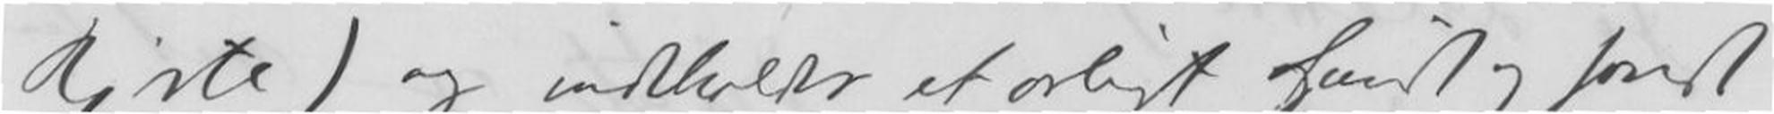Hjerte) og indholder et ærligt sundt og sandt
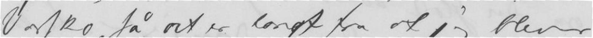Varsko så det er langt fra at jeg bliver
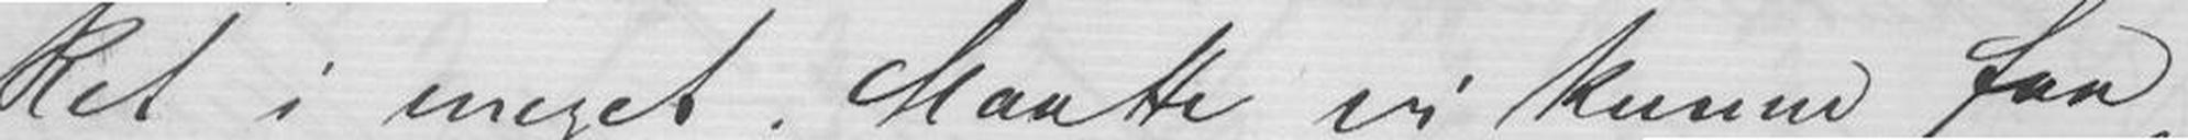Ret i meget. Maatte vi kunne faa
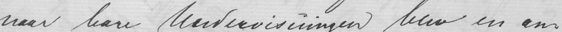naar bare Undervisningen blev en an-
